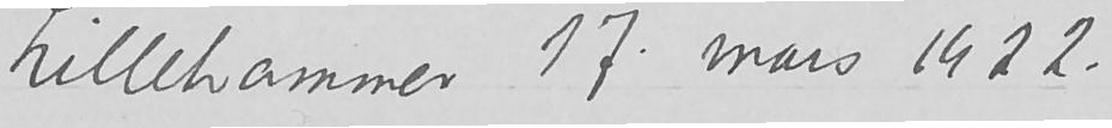Lillehammer 17. mars 1922
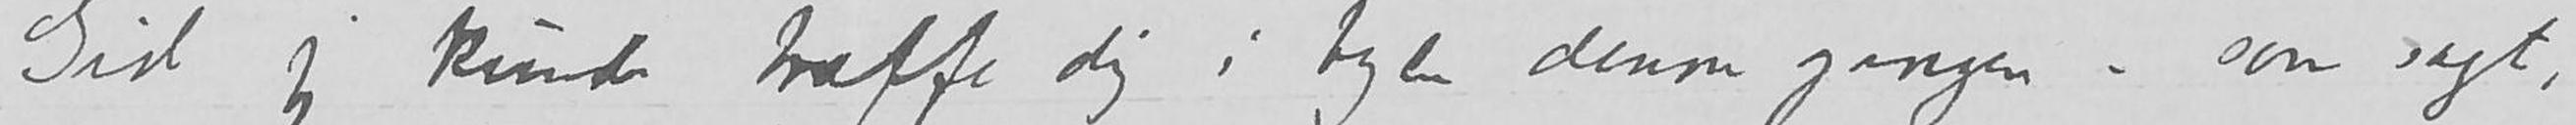Gid jeg kunde træffe dig i byen denne gangen – som sagt, skrive
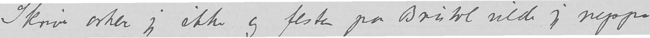Skrive orker jeg ikke og festen på Bristol vilde jeg neppe
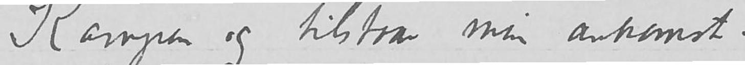Kampen og tilstaar min ankomst.
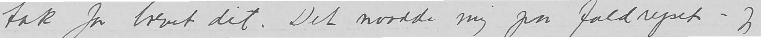tak for brevet dit.  Det naadde mig paa faldrepet – jeg
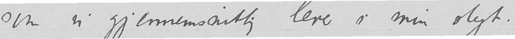som vi gjennemsnitlig lever i min slegt.
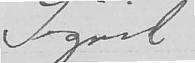Sigrid
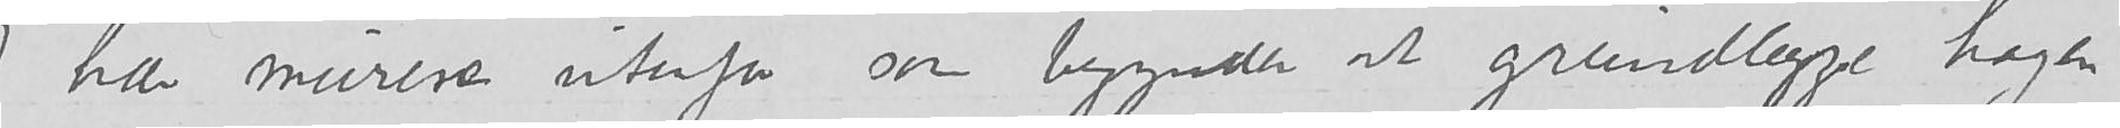Jeg har murere utenfor som begynder at grundlegge hagen
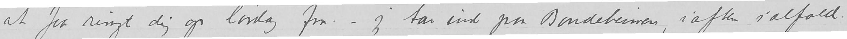at faa ringt dig op lørdag frm - jeg tar ind paa Bondeheimen, i aften i alfald.
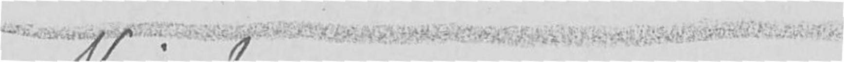Nu begynder jeg at merke at jeg har tat op paa forskud av de 80-90 aarerne
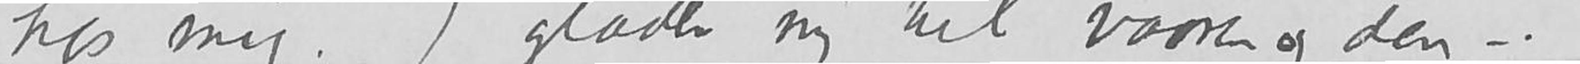hos mig. Jeg glæder mig til vaaren og den –.
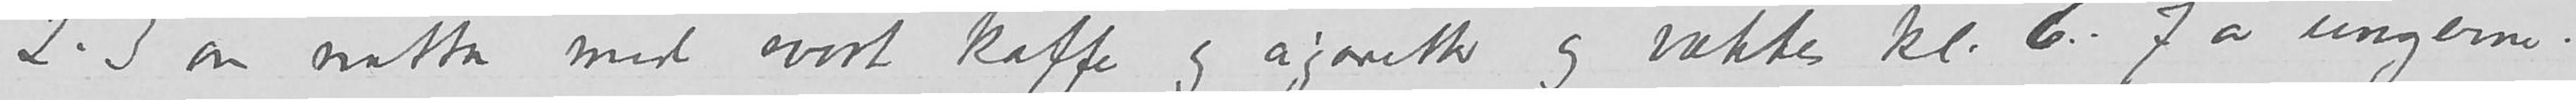2-3 om natten med svart kaffe og sigaretter og vækkes kl. 6-7 av ungerne.
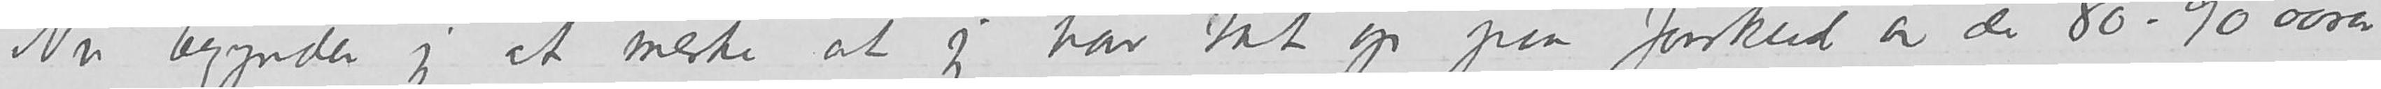Nu begynder jeg at merke at jeg har tat op paa forskud av de 80-90 aarer
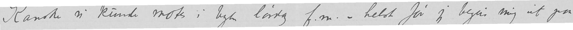Kanske vi kunde møtes i byen lørdag f.m. – helst før jeg begir mig ut paa
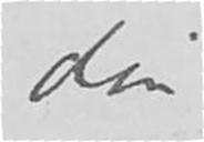din
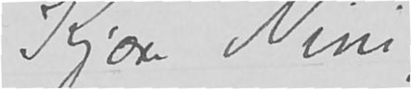Kjære Nini
